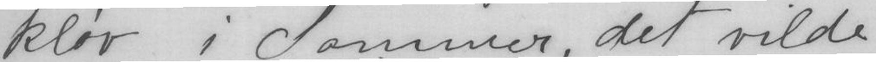kløv i Sommer, det vilde
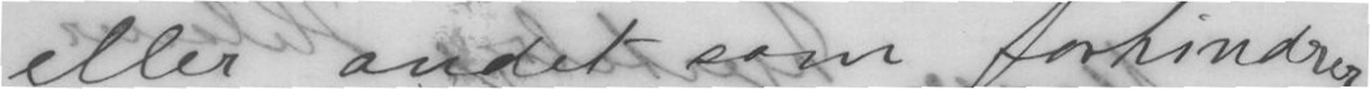eller andet som forhindrer
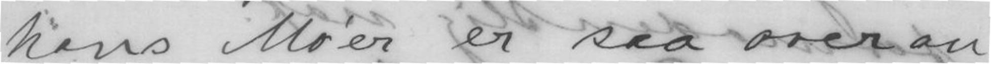hans Moér er saa overan
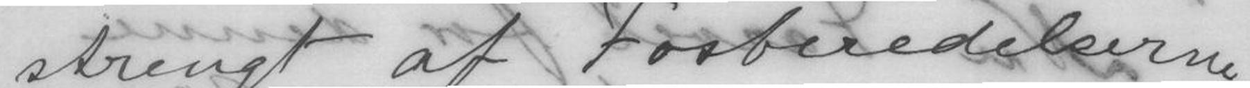strengt af Forberedelserne
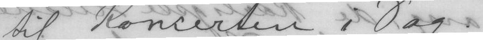til Koncerten i Dag.
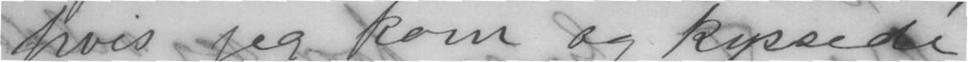hvis jeg kom og kyssede
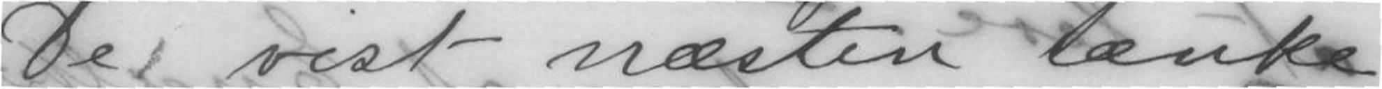De vist næsten tænke
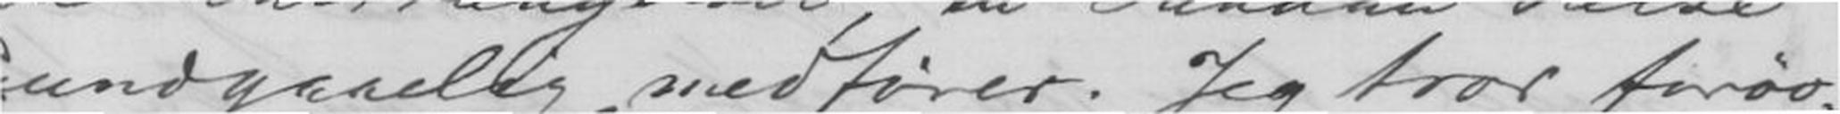uundgaaelig medfører. Jeg tror forøv-
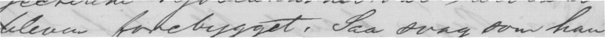bleven forebygget. Saa svag som han
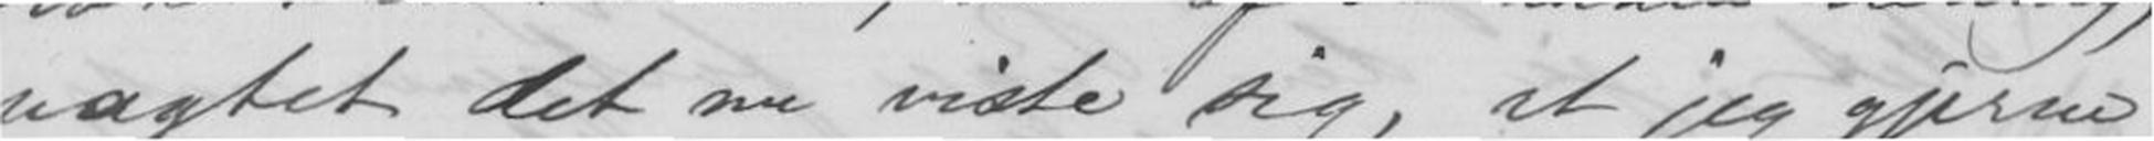uagtet det nu viste sig, at jeg gjerne
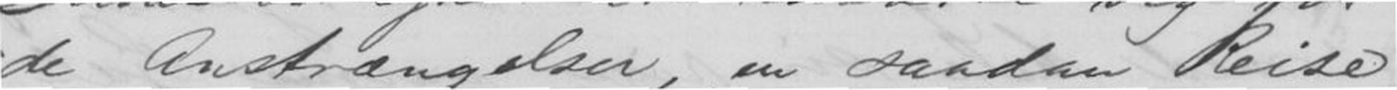de Anstrængelser, en saadan Reise
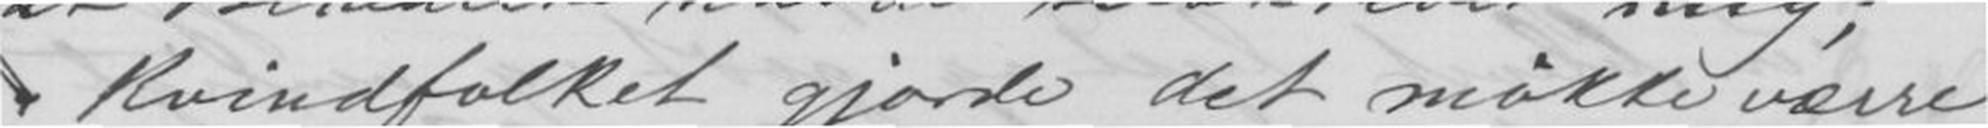Kvindfolket gjorde det møkke værre
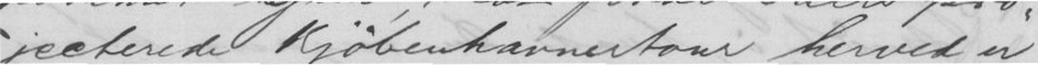jecterede Kjøbenhavnertour herved er
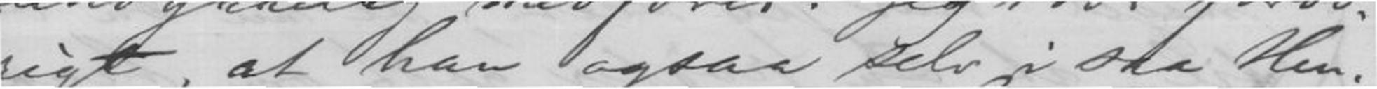rigt, at han ogsaa selv i saa Hen-
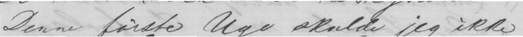Denne første Uge skulde jeg ikke
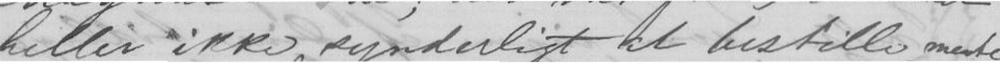heller ikke synderligt at bestille, mente
